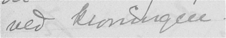ved kroningen.
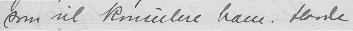som vil konsulere ham. Havde
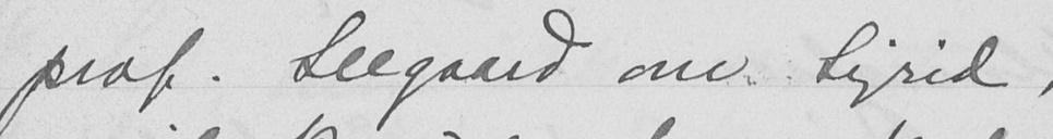prof. Leegaard om Sigrid,
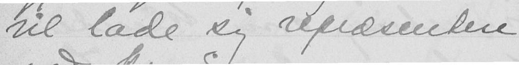vil lade sig repræsentere
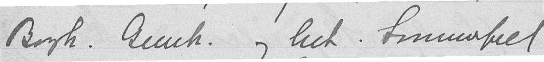Borgh. Gunh. og lnt. Sommerfelt
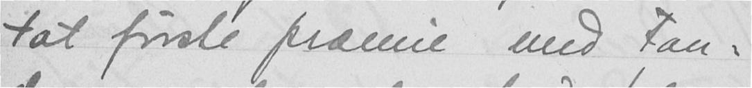tat første præmie med Fan-
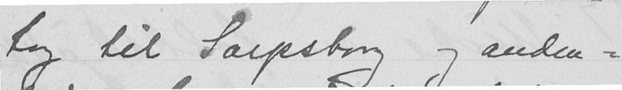tog til Sarpsborg og anden-
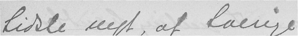Sidste nyt, at Sverige
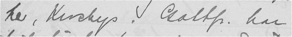her, Krosbys. Gottfr. har
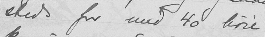steds for med 40 høie
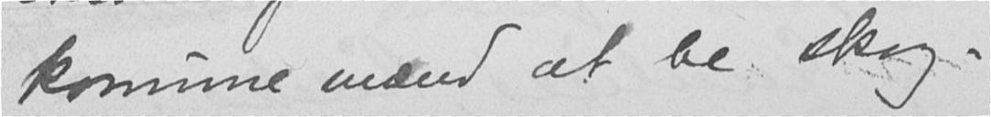komune mænd at be skog-
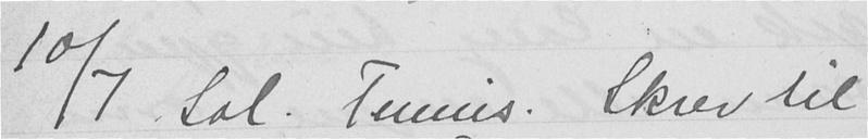10/7 Sol. Tennis. Skrev til
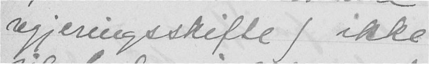regjeringsskifte) ikke
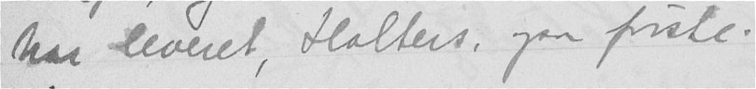har leveret, Halters, ogsaa første.
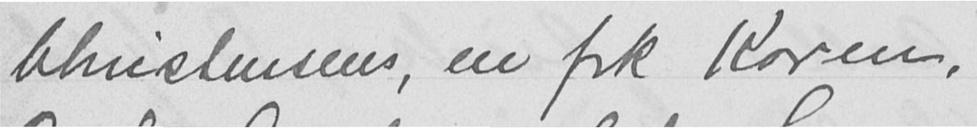Christensens, en frk Koren,
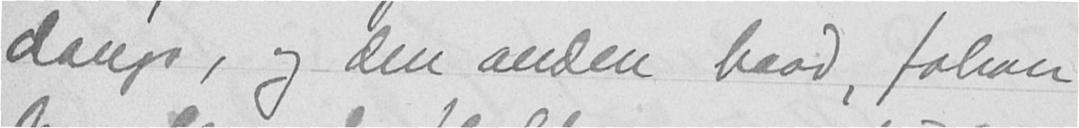dango, og den anden baad, Johan
